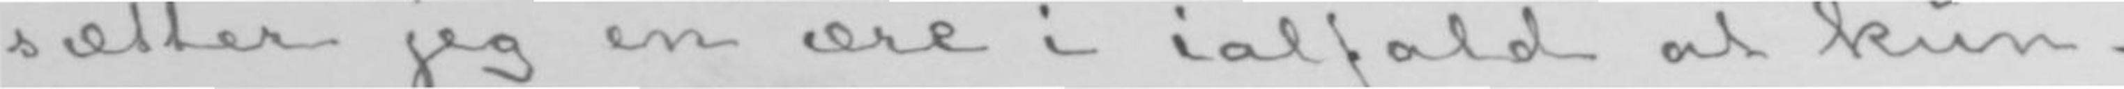sætter jeg en ære i ialfald at kun-
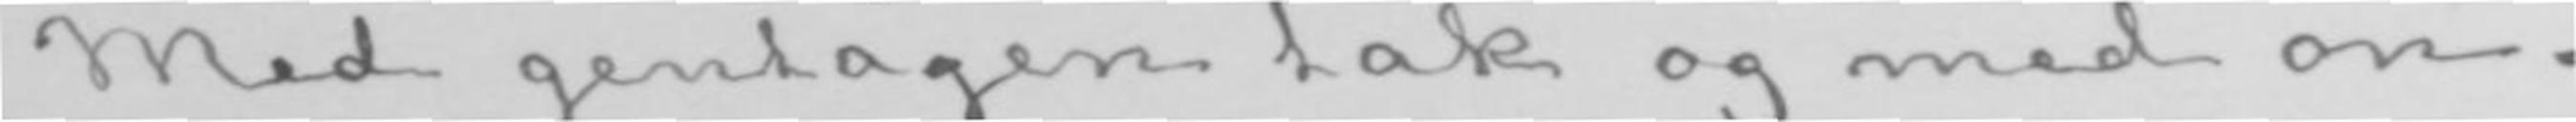Med gentagen tak og med øn-
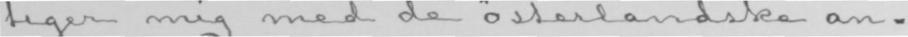tiger mig med de østerlandske an-
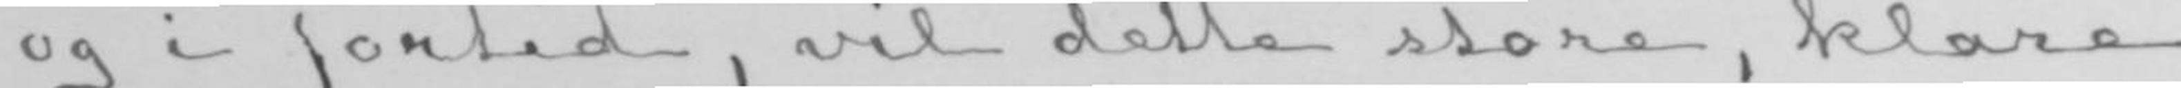og i fortid, vil dette store, klare
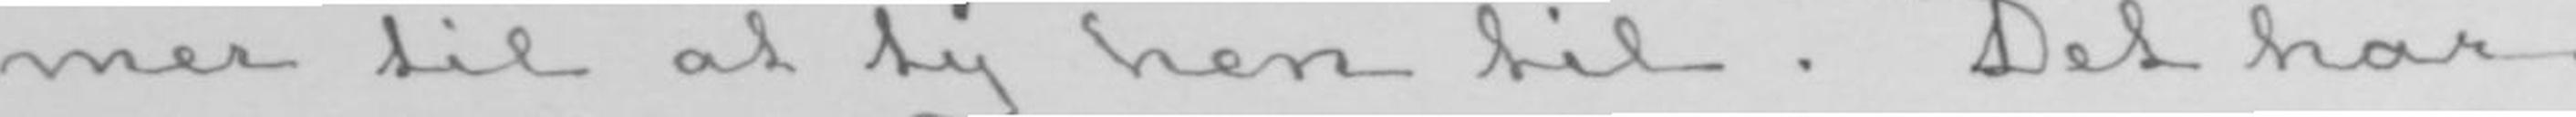mer til at ty hen til. Det har
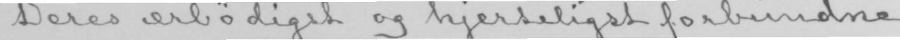Deres ærbødigst og hjerteligst forbundne
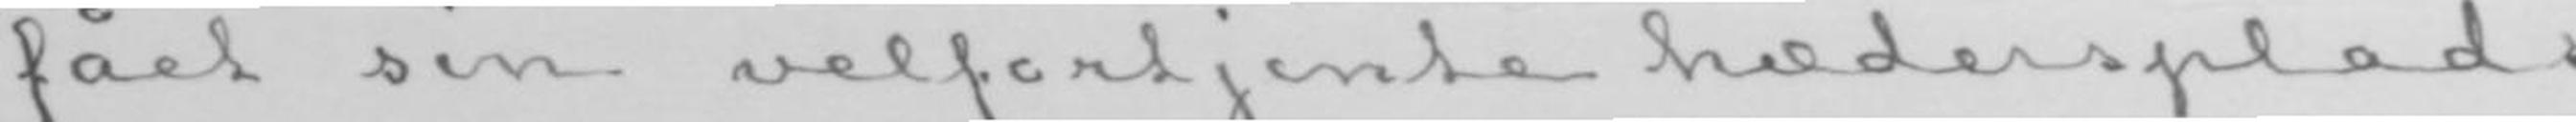fået sin velfortjente hædersplads
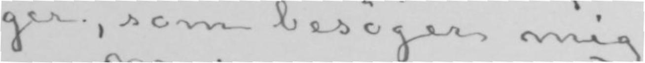ger, som besøger mig.
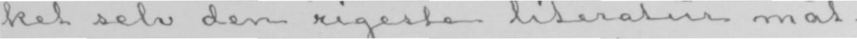ket selv den rigeste literatur måt-
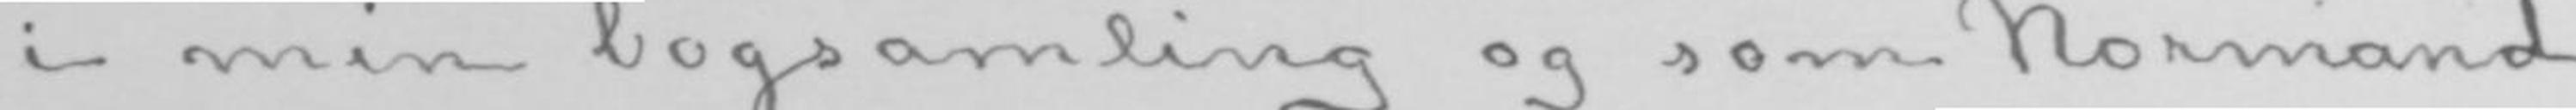i min bogsamling og som Normand
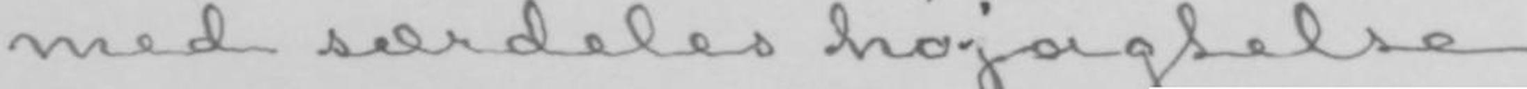med særdeles højagtelse
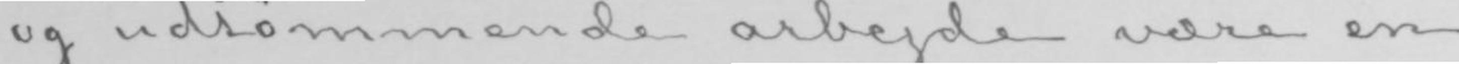og udtømmende arbejde være en
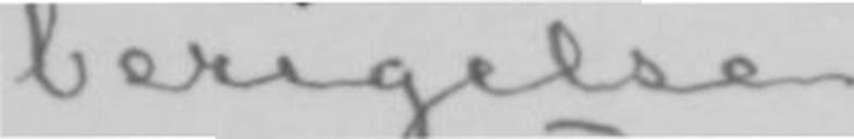berigelse.
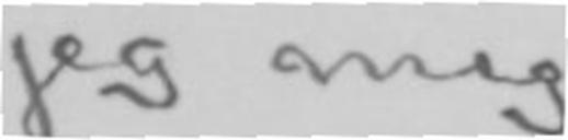jeg mig
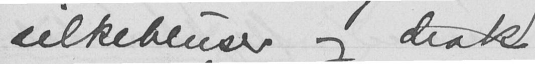silkebluser og drak
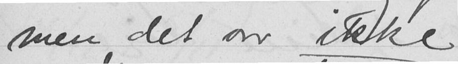men det var ikke
